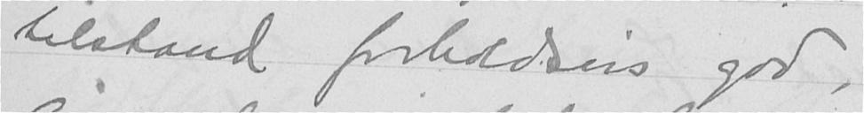tilstand forholdsvis god,
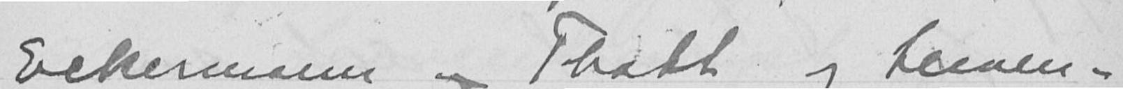Eckermann og Thott og Lewen-
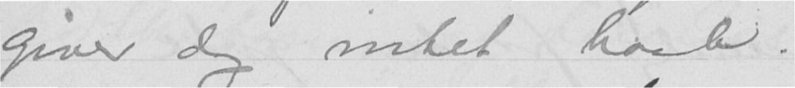giver dog intet haab.
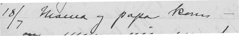18/7 Mama og papa kom -
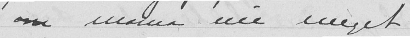om mama nu meget
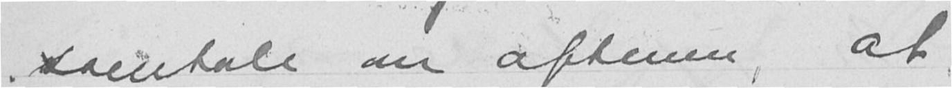samtale om aftenen, at
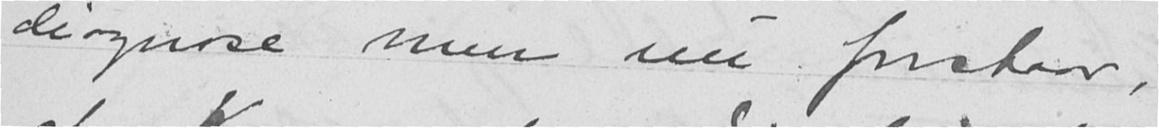diagnose men nu forstaar,
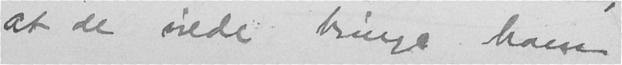at de vilde bringe ham
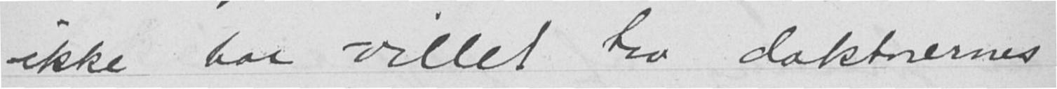ikke har villet tro doktorernes
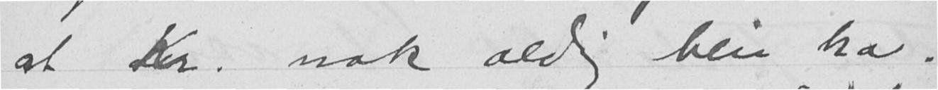at Kr. nok aldrig blir bra.
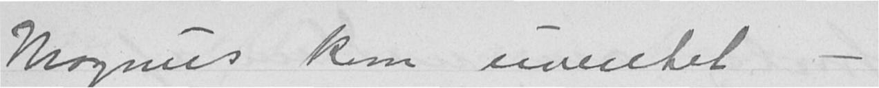Magnus kom uventet -
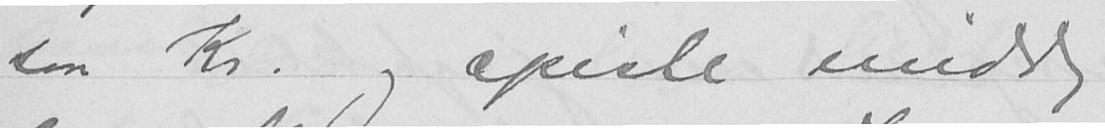saa Kr. og spiste middag
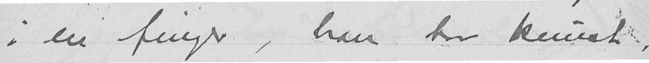i en finger, han har knust,
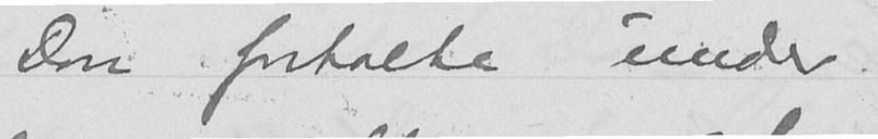Don fortalte under
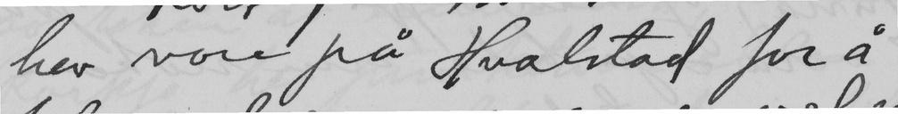hev vore på Hvalstad for å
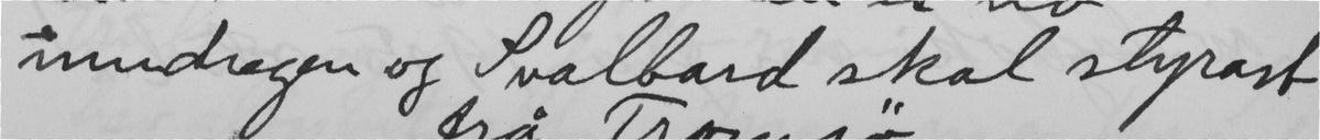umdregen og Svalbard skal styrast
